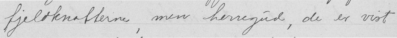fjeldknatterne, men herregud, de er vist
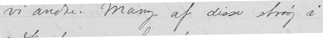vi andre. Mange af disse strøg i
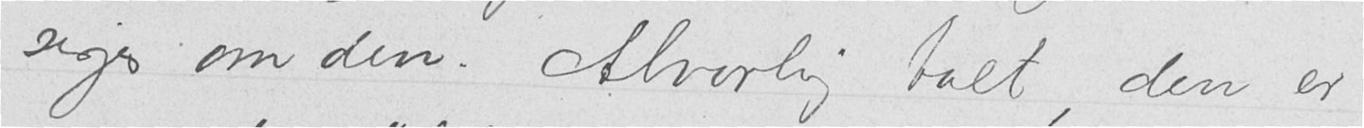siges om den. Alvorlig talt, den er
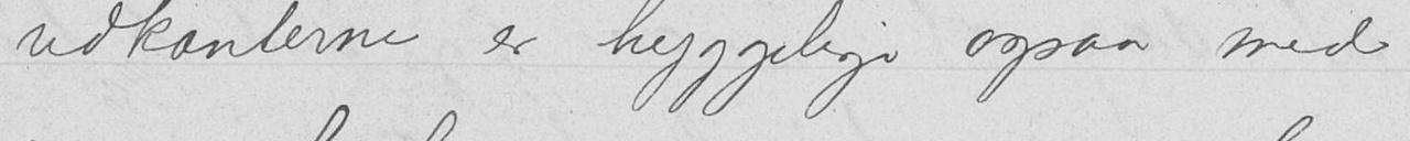udkanterne er hyggelige ogsaa med
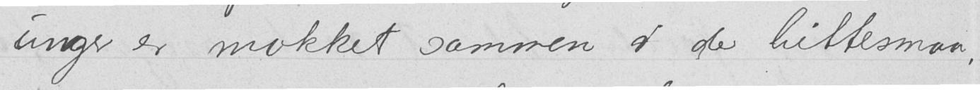unger er mokket sammet i de bittesmaa,
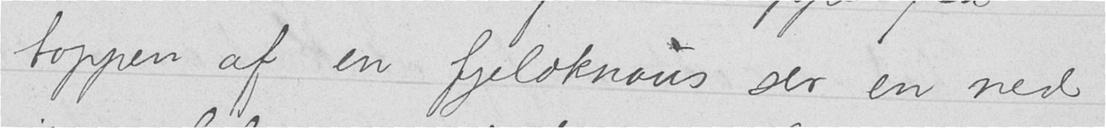toppen af en fjeldknaus ser en ned
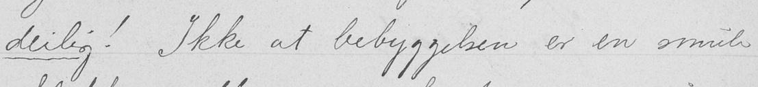deilig! Ikke at bebyggelsen er en smule
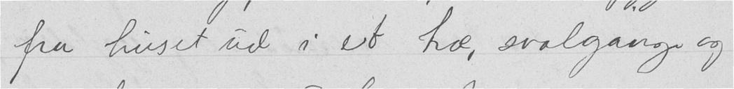fra huset ud i et træ, svalganger og
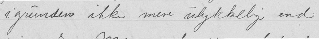igrunden ikke mere ulykkelige end
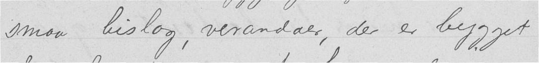smaa bislag, verandaer, der er bygget
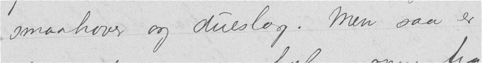smaahaver og dueslag. Men saa
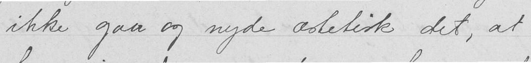ikke gaa og nyde æstetisk det, at
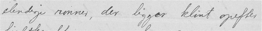elendige rønner, der ligger klint opefter
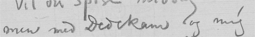men med Dedekam og mig
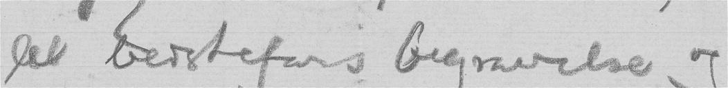le bedstefars begravelse og
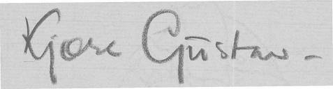Kjære Gustav -
